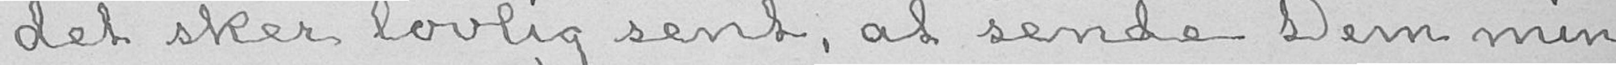det sker lovlig sent, at sende Dem min
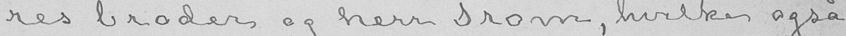res broder og herr Prom, hvilke også
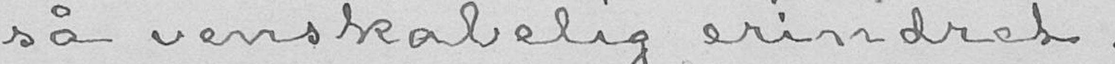så venskabelig erindret.
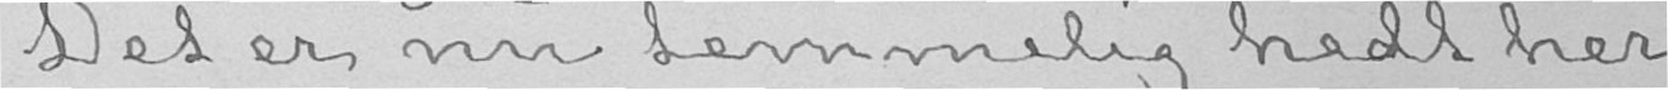Det er nu temmelig hedt her
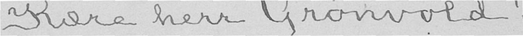Kære herr Grønvold!
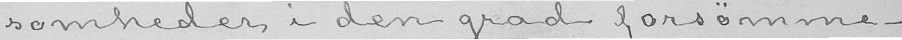somheder i den grad forsømme-
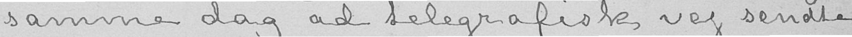samme dag ad telegrafisk vej sendte
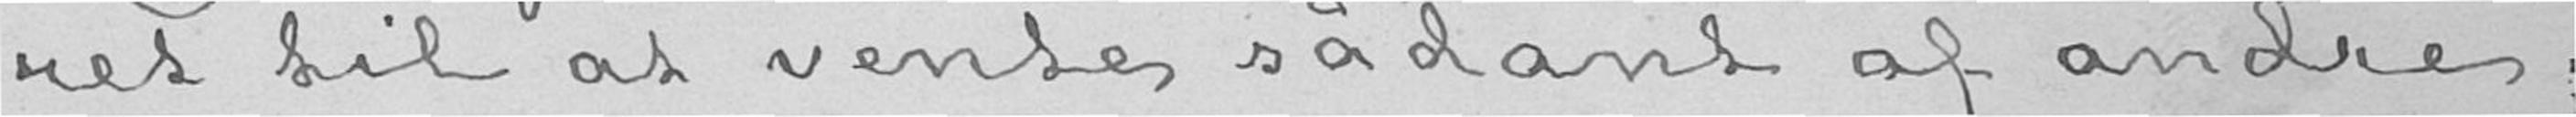ret til at vente sådant af andre;
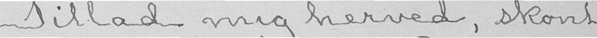Tillad mig herved, skønt
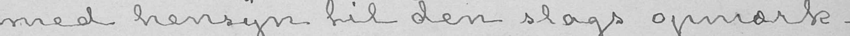med hensyn til den slags opmærk-
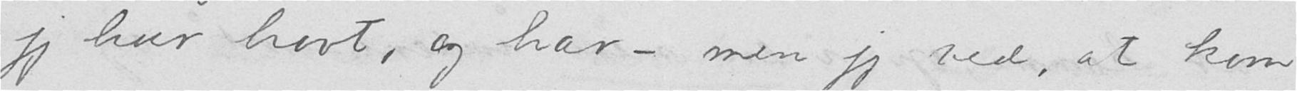jeg har havt, og har - men jeg ved, at kom
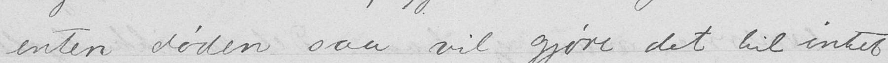enten døden saa vil gjøre det til intet
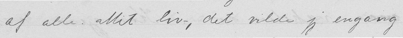af alle. Mit liv, det vilde jeg engang
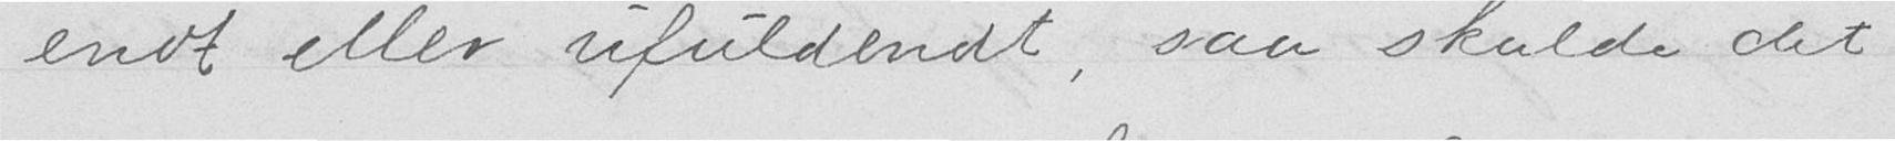endt eller ufuldendt, saa skulde det
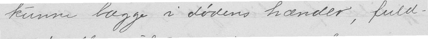kunne lægge i dødens hænder, fuld-
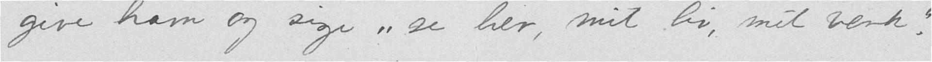give ham og sige "se her, mit liv, mit verk."
